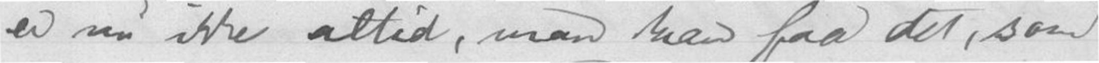er nu ikke altid, man kan faa det, som
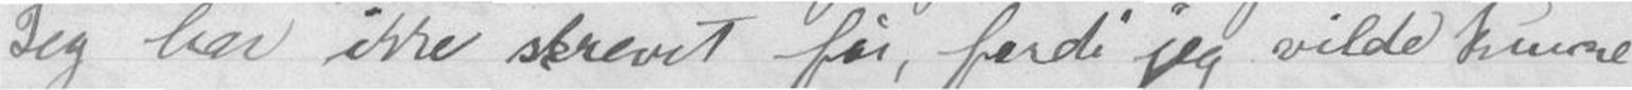Jeg har ikke skrevet før, fordi jeg vilde kunne
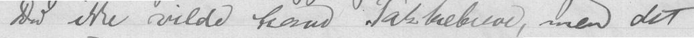Du ikke vilde have Takkebreve, men det
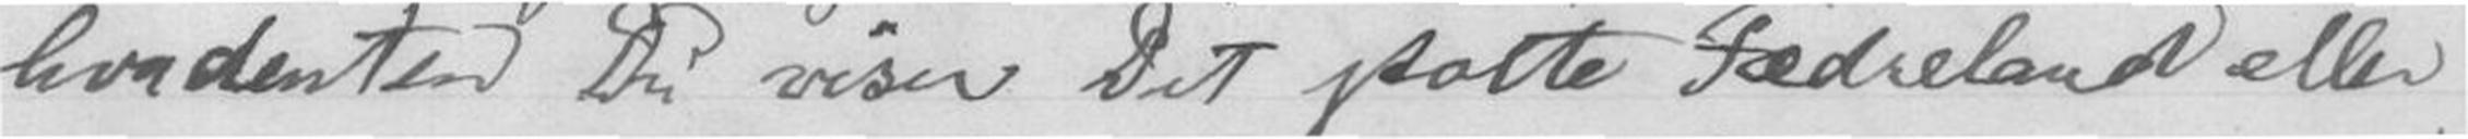hvadenten Du viser Dit stolte Fædreland eller
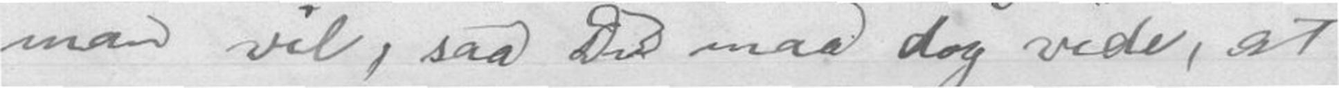man vil, saa Du maa dog vide, at
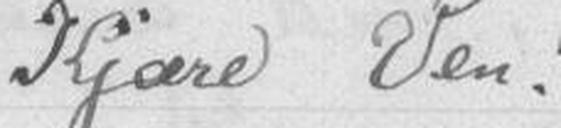Kjære Ven!
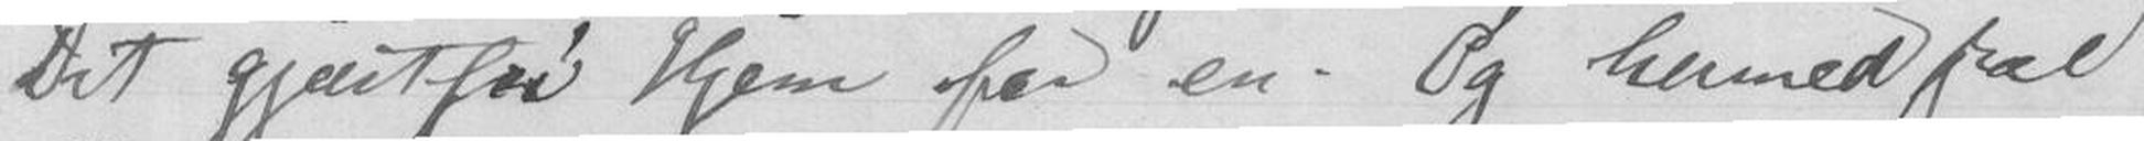Dit gjæstfri Hjem for en. Og hermed skal
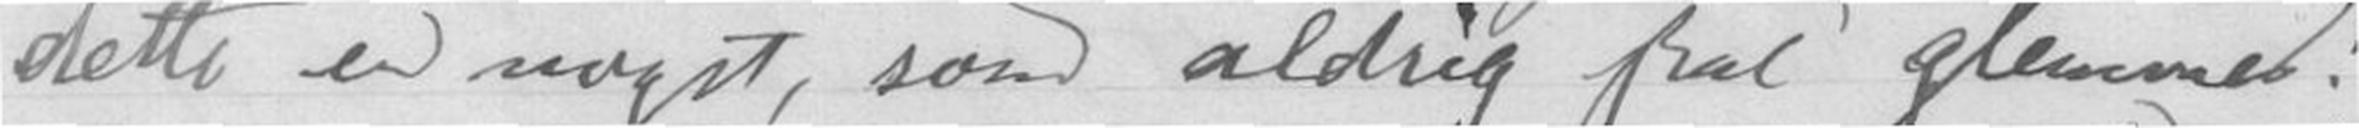dette er noget, som aldrig skal glemmes!
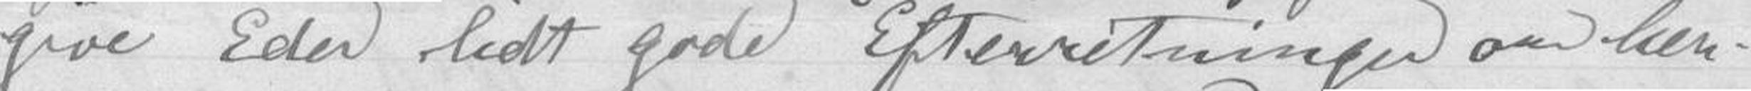give Eder lidt gode Efterretninger om hen-
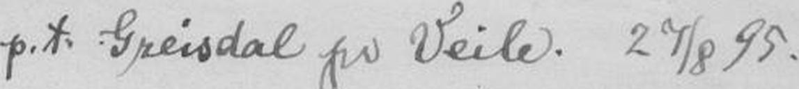p.t. Greisdal pr Veile. 27/8 95.
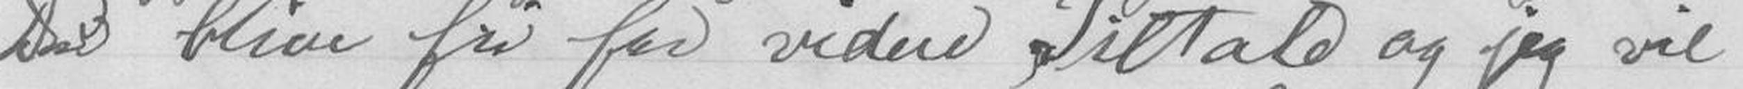Du blive fri for videre Tiltale og jeg vil
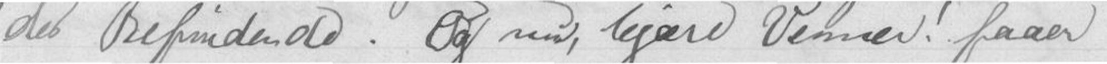des Befindende. Og nu, kjære Venner! faaer
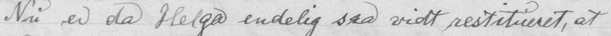Nu er da Helga endelig saa vidt restitueret, at
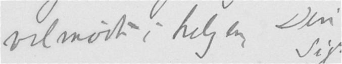velmødt i helgen Din
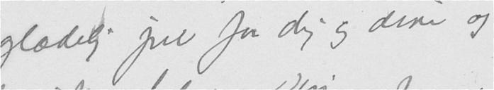glædelig jul for dig og dine og
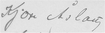Kjære Åslaug
